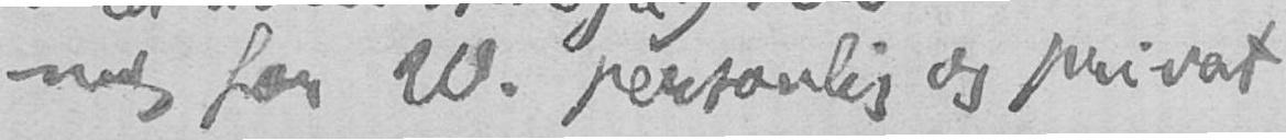mig for W. personlig og privat
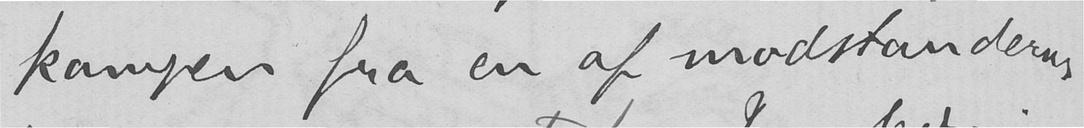kampen fra en af modstandernes
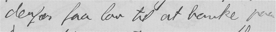derfor faa lov til at banke paa
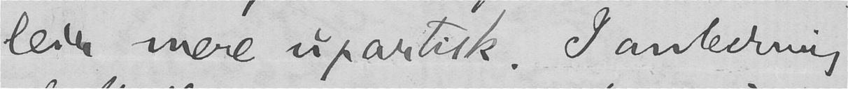leir mere upartisk. I anledning
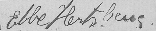Ebbe Hertzberg.
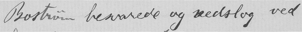Bostrøm besvarede og nedslog ved
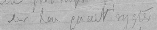Der har gaaet rygter
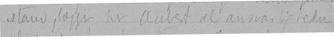istand, lægger hr Aubert al ansvarligheden
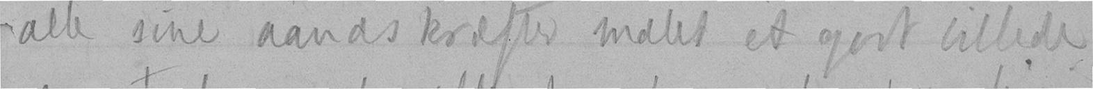alle sine aandskræfter malet et godt billede
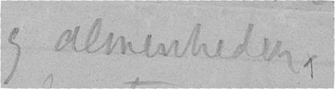og almenheden,
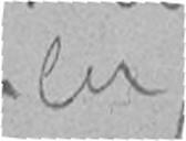en
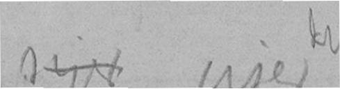siger viser
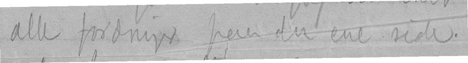alle fordringer paa den ene side.
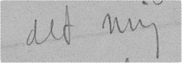det mig
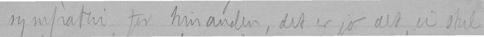sympathi for hinanden, det er jo det vi skul-
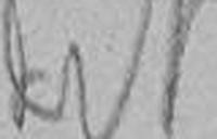det
